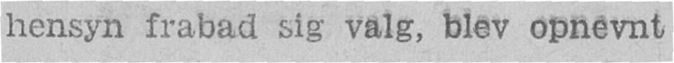hensyn frabad sig valg, blev opnevnt
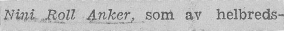Nini Roll Anker, som av helbreds-
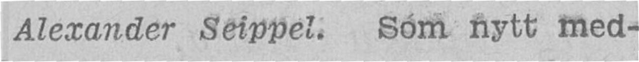Alexander Seippel. Som nytt med-
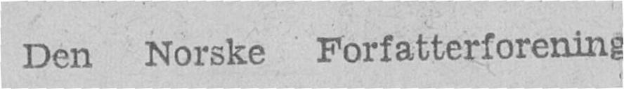Den Norske Forfatterforening
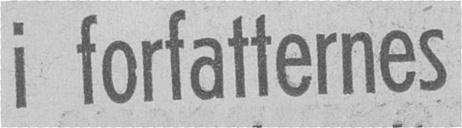i forfatternes
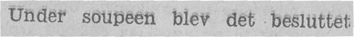Under soupeen blev det besluttet
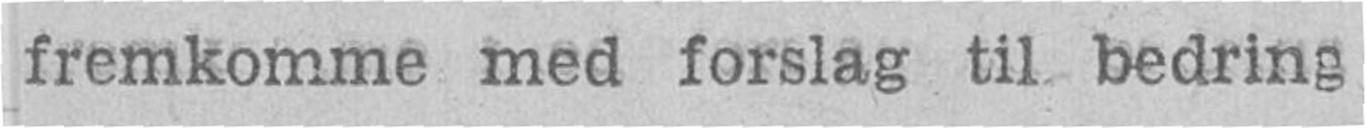fremkomme med forslag til. bedring
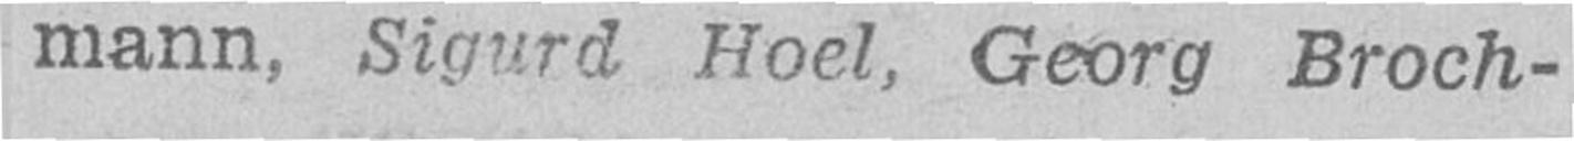mann, Sigurd Hoel, Georg Broch-
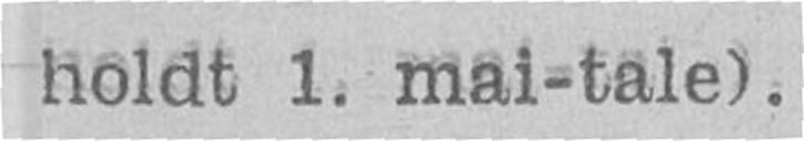holdt 1. mai-tale).
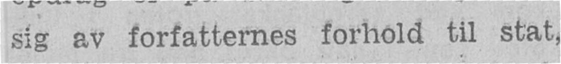sig av forfatternes forhold til stat,
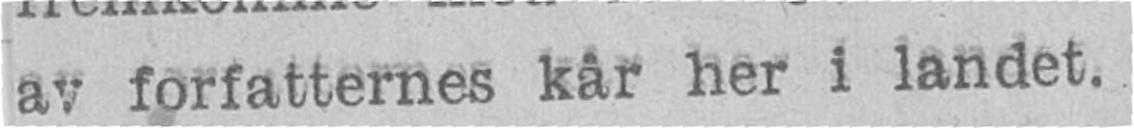av forfatternes kår her i landet.
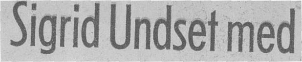Sigrid Undset med
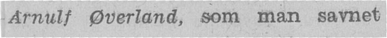Arnulf Øverland, som man savnet
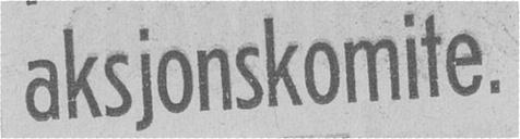aksjonskomite.
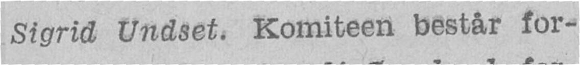Sigrid Undset. Komiteen består for-
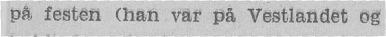på festen (han var på Vestlandet og
